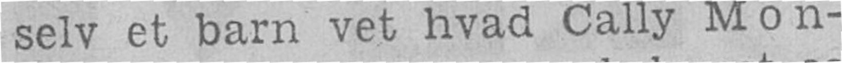selv et barn vet hvad Cally Mon-
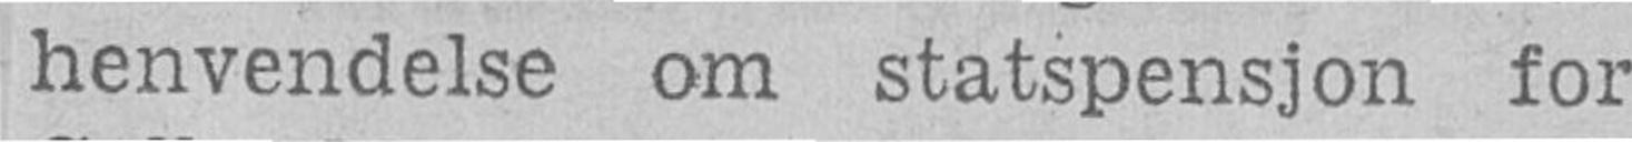henvendelse om statspensjon for
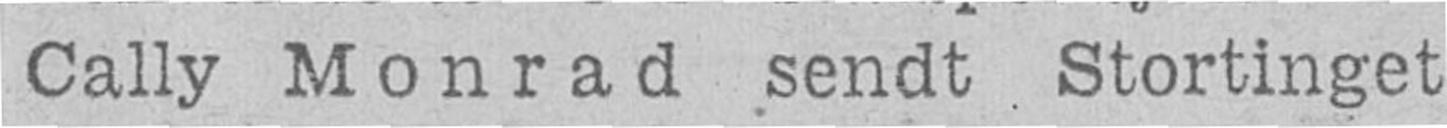Cally Monrad sendt Stortinget
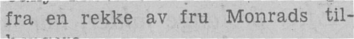fra en rekke av fru Monrads til-
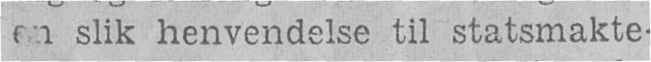en slik henvendelse til statsmakte-
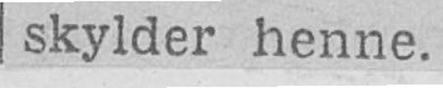skylder henne.
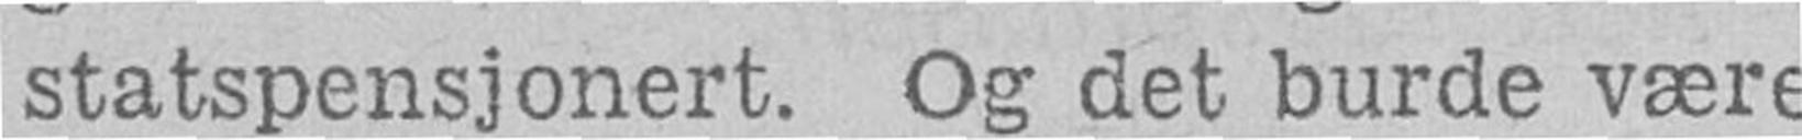statspensjonert. Og det burde være
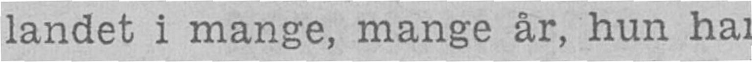landet i mange, mange år, hun har
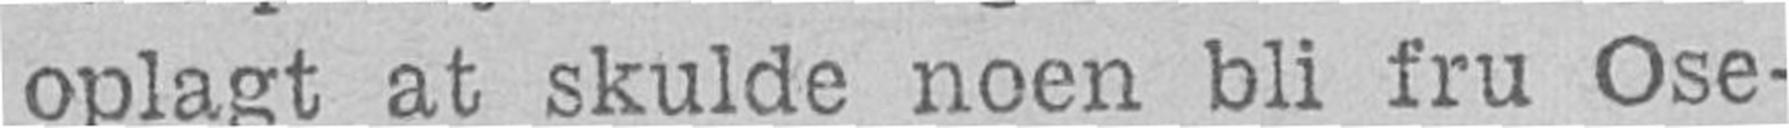oplagt at skulde noen bli fru Ose-
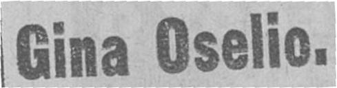Gina Oselio.
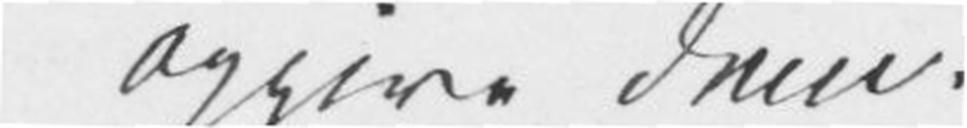opgive dem.
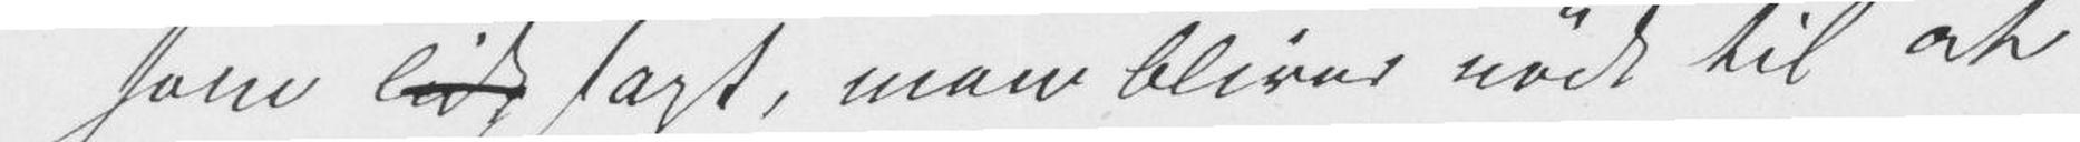som sagt, man bliver nødt til at
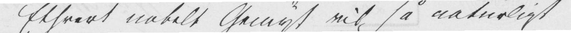Ethvert nobelt Gemyt vil så naturligt
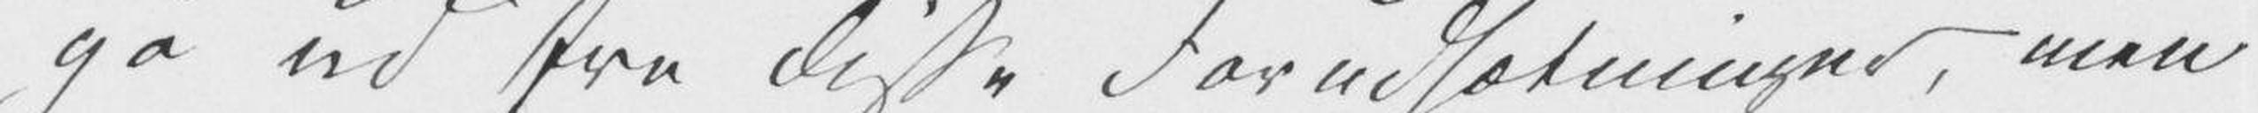gå ud fra disse Forudsætninger, men
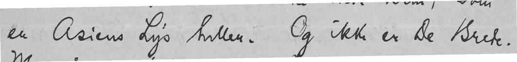er Asiens Lys heller. Og ikke er De Brede.
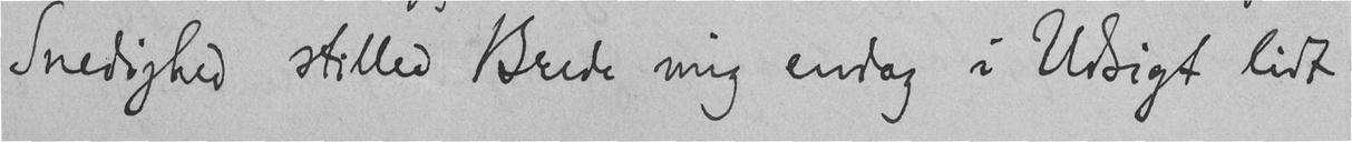Snedighed stilled Brede mig endog i Udsigt lidt
